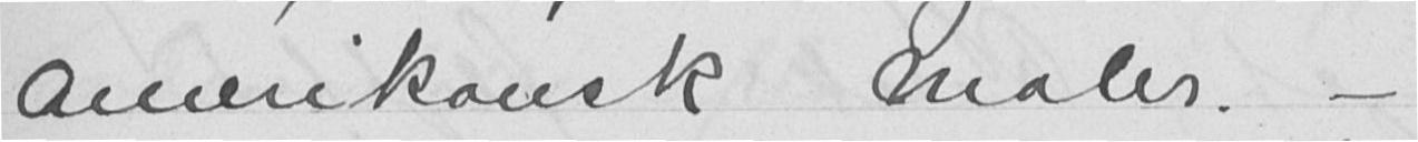amerikansk maler. -
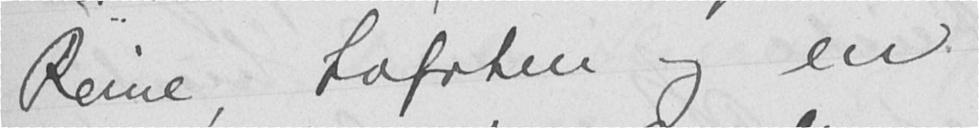Reine, Lofoten og en
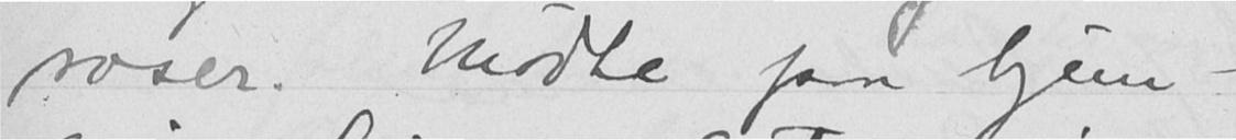roser. Mødte paa hjem-
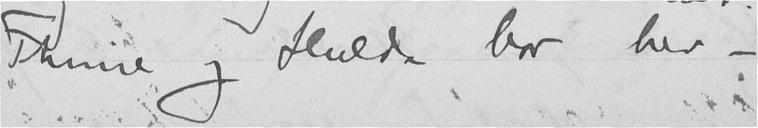Thrine og Hulda bor her -
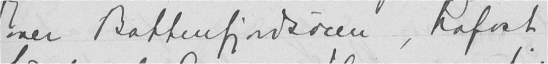over Battenfjordsøren, trofast
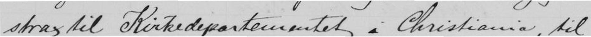strax til Kirkedepartementet i Christiania, til
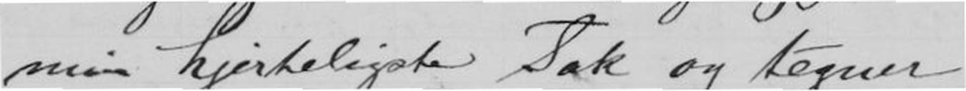min hjerteligst Tak og tegner
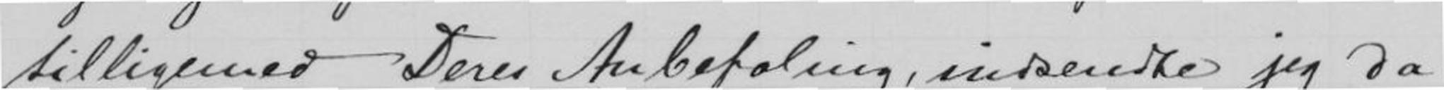tilligemed Deres Anbefaling, indsendte jeg da
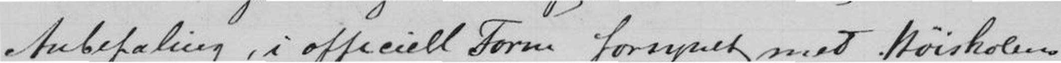Anbefaling, i officiell Form forsynet med Høiskolens
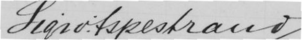Sigw. Aspestrand
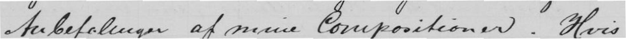Anbefaling af mine Compositioner. Hvis
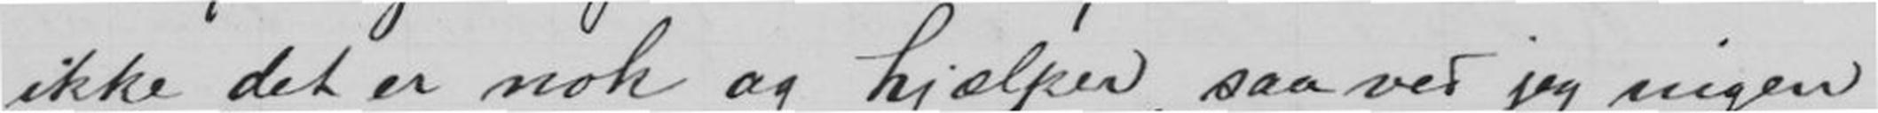ikke det er nok og hjælper, saa ved jeg ingen
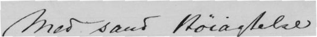Med sand Høiagtelse
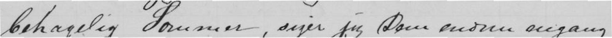behagelig Sommer, siger jeg Dem endnu engang
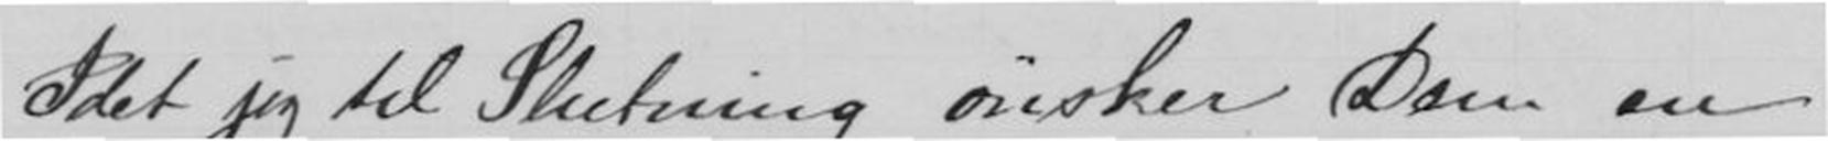Det jeg til Slutning ønsker Dem en
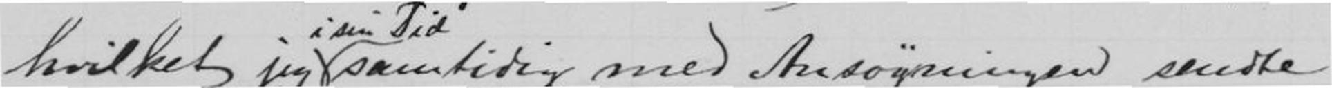hvilket jeg samtidig med Ansøgning sendte
